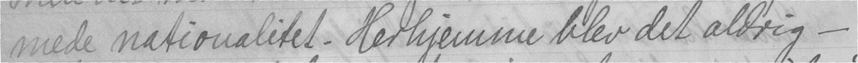mede nationalitet. Herhjemme blev det aldrig -
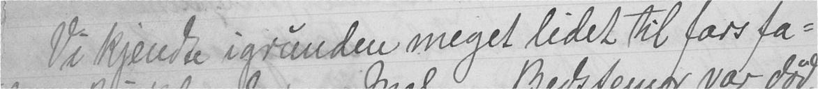Vi kjendte igrunden meget lidet til fars fa-
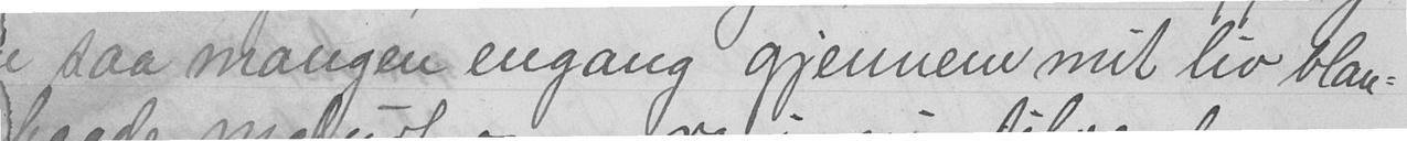saa mangen engang gjennem mit liv blan-
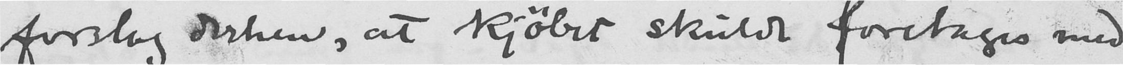forslag derhen, at kjøbet skulde foretages med
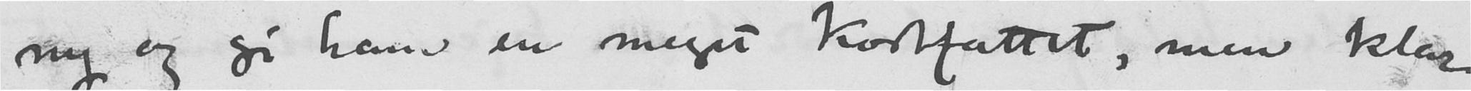ny og gi ham en meget kortfattet, men klar
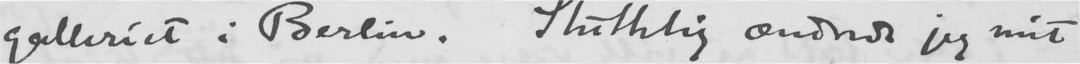galleriet i Berlin. Sluttelig ændrede jeg mit
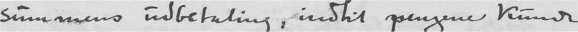summens udbetaling, indtil pengene kunde
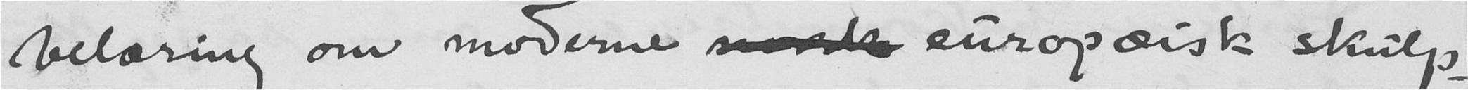belæring om moderne norsk europeisk skulp-
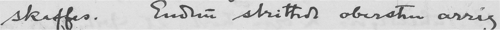skaffes. Endnu strittede obersten arrig
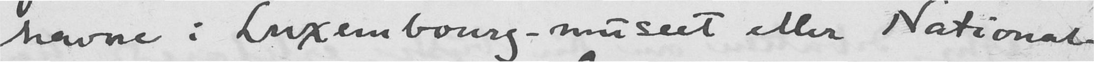havne i Luxembourg-museet eller National-
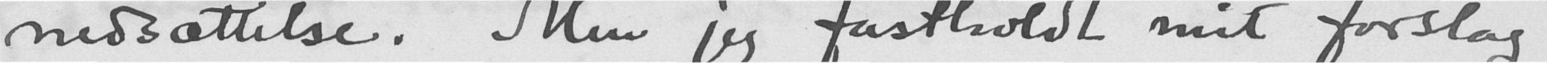nedsættelse. Men jeg fastholdt mit forslag
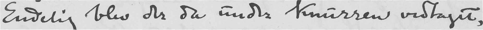Endelig blev der da under knurren vedtaget,
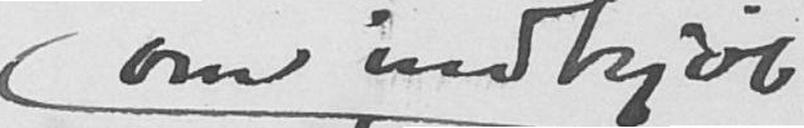om indkjøb
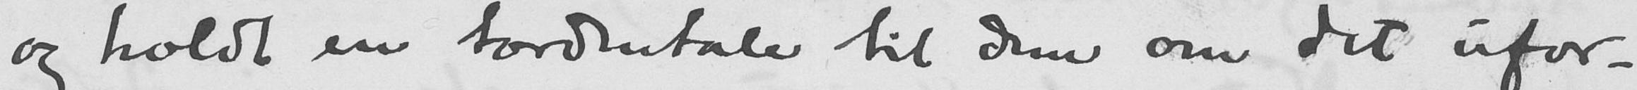og holdt en tordentale til dem om det ufor-
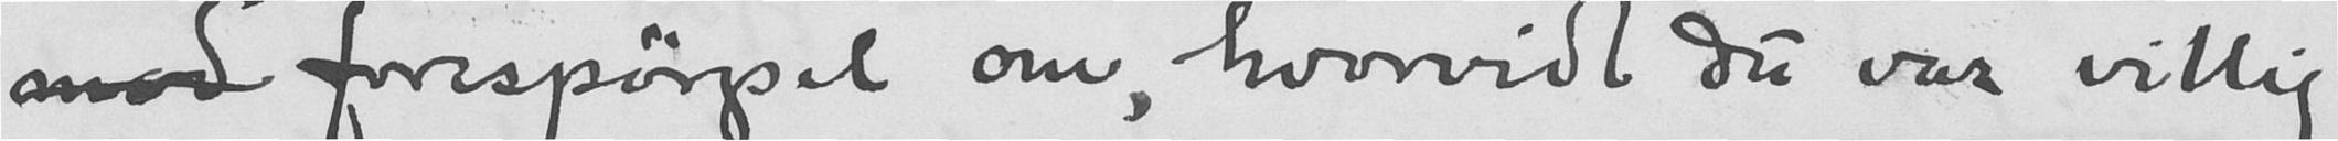mod forespørgsel om, hvorvidt du var villig
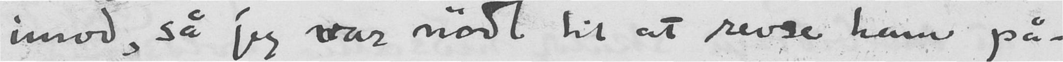imod, så jeg var nødt til at revse ham på
